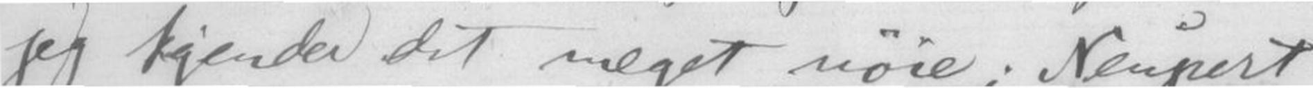jeg kjender det meget nøie; Neupert
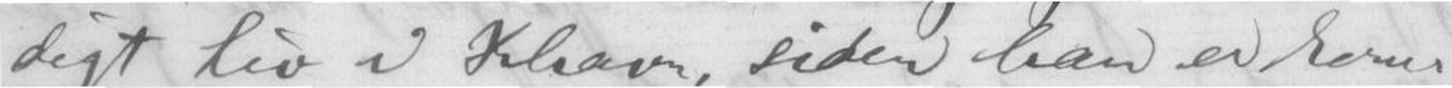digt Liv i Khavn, siden han kom-
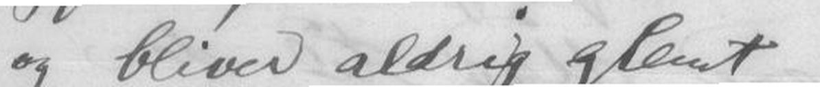og bliver aldrig glemt.
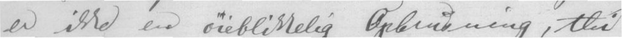er ikke en øieblikkelig Opbrusning, thi
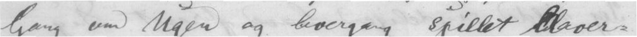Gang om Ugen og hver gang spillet Claver-
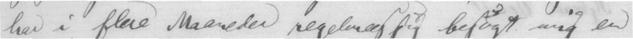har i flere Maaneder regelmæssig besøgt mig en
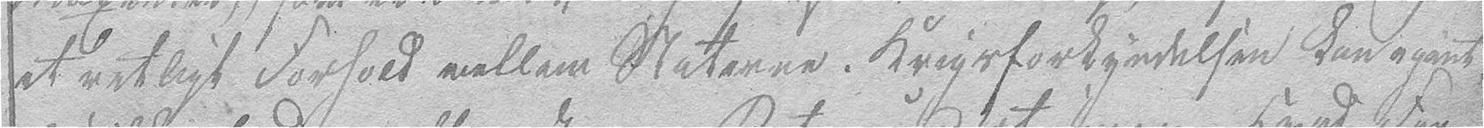et retligt Forhold mellem Staterne. Krigsforkyndelsen kan egent-
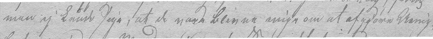man ey kunde sige at de vare blevne enige om at afgjøre Uenig-
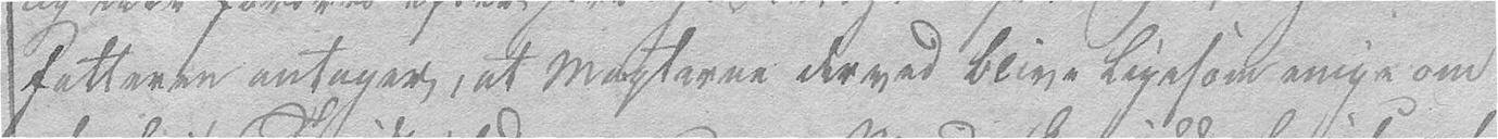fatteren antager, at Magterne derved blive ligesom enige om
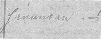hinanden. –
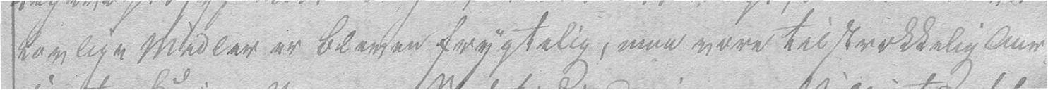lovlige Midler er bleven frygtelig, maa være tilstrækkelig Aar-
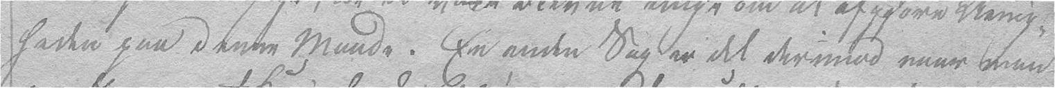heden paa denne Maade. En anden Sag er det derimod naar man
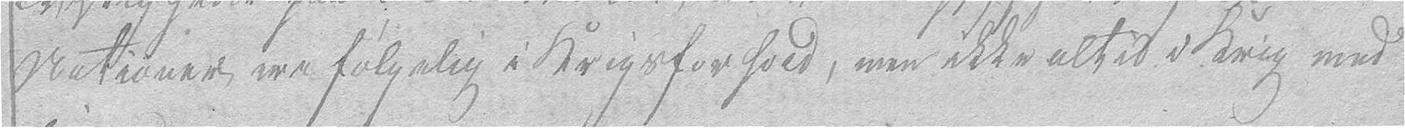Nationer ere følgelig i Krigsforhold, men ikke altid i Krig med
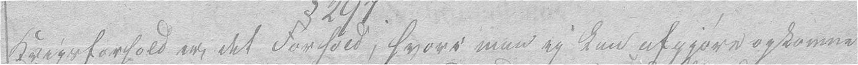Krigsforhold er det Forhold, hvori man ey kan afgjøre opkomne
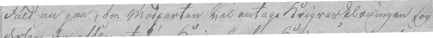Fald an paa, om Modparten vil antage Krigserklæringen, og
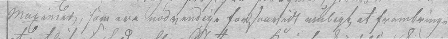Maximer, som ere nødvendige forsaavidt muligt at frembringe
